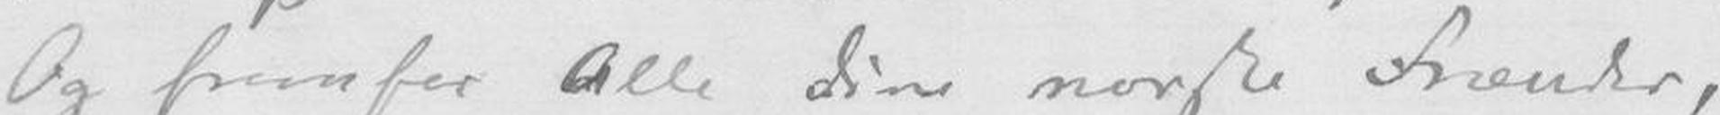Og fremfor Alle dine norske Frænder,
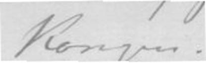Kongen
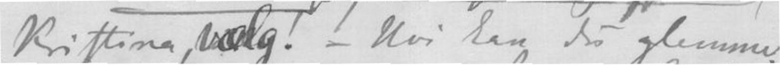Kristina, vælg! - Hvi kan du glemme,
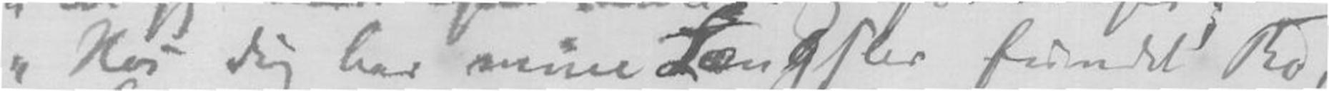"Hod dig har mine Længsler fundet Ro,
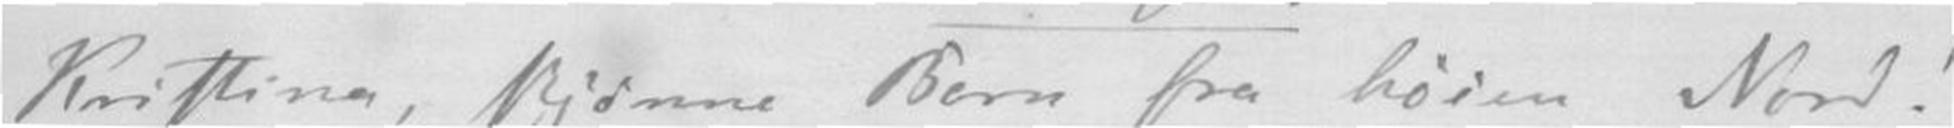Kristina, skjønne Born fra høien Nord!
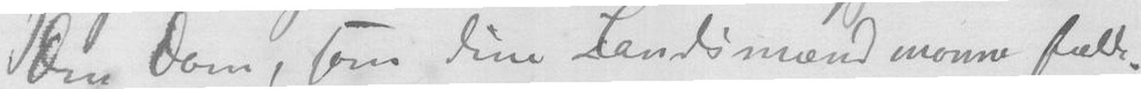Din Dom, som dine Landsmænd monne fælde.
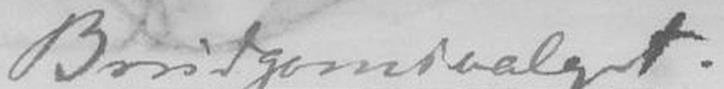Brudgomsvalget.
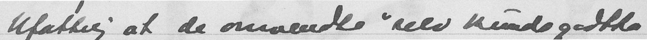Ufattelig at de omvendte selv kunde godtta
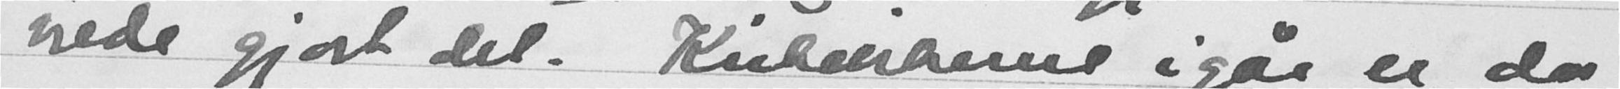vilde gjort det. Kritikkerne i går er da
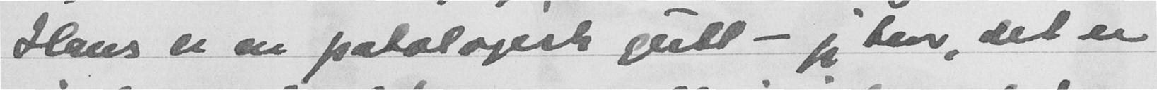Hans er en patologisk gutt -  jeg tror det er
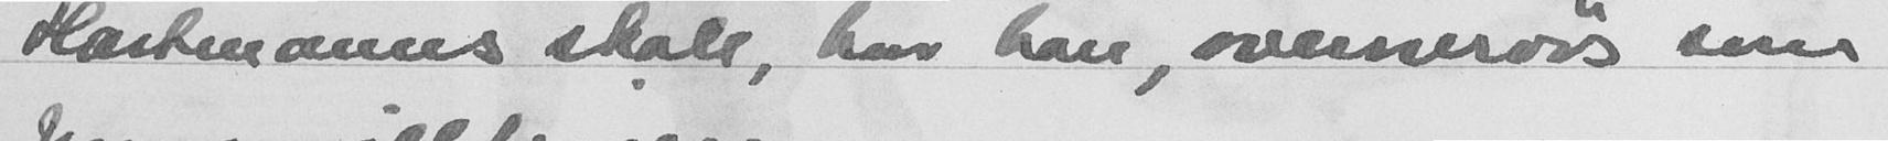Hartmanns skole, hvor han, overnervøs som
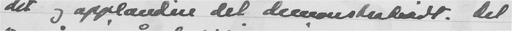det og applaudere det decnstrabudt. Det
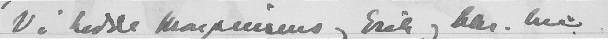Vi hadde kronprinsens og Erik og Chr. her
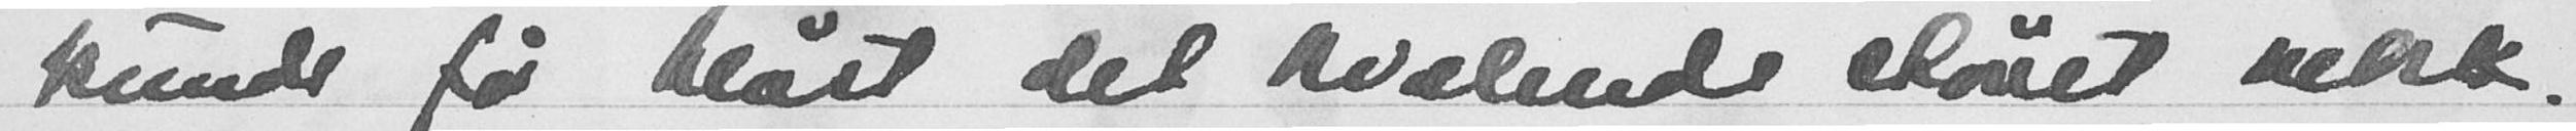kunde få blåst det kvælende støvet vekk!
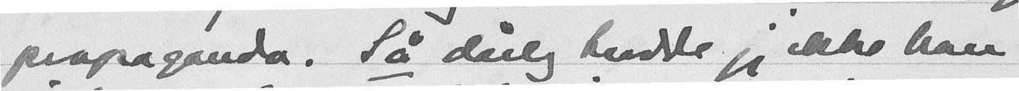propaganda. Så dårlig trodde jeg ikke han
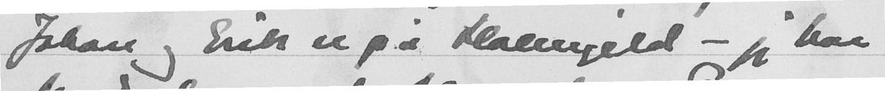Johan og Erik er på Holmgild - jeg har
